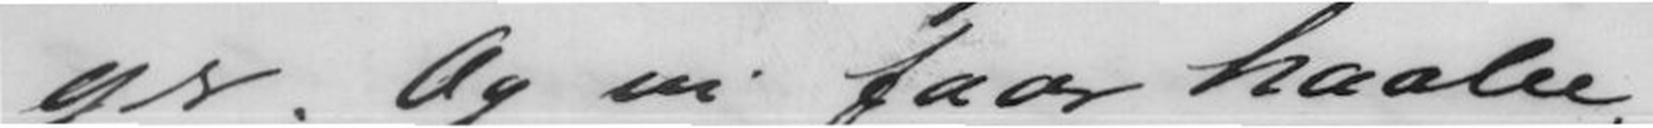ger. Og vi faar haabe,
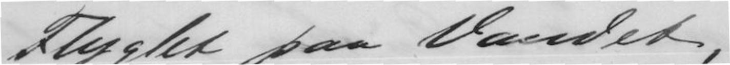Flyglet paa Vandet,
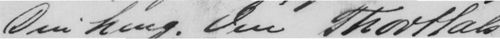Din heng. Ven Thor Hals
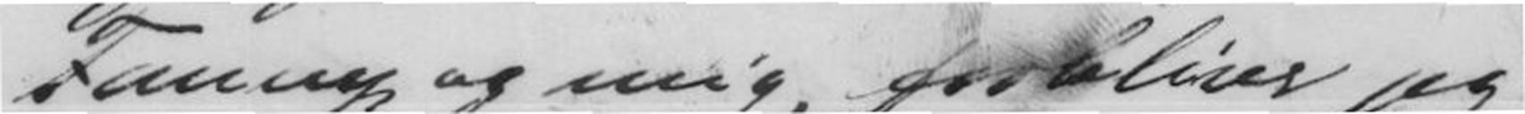Fanny og mig, forbliver jeg
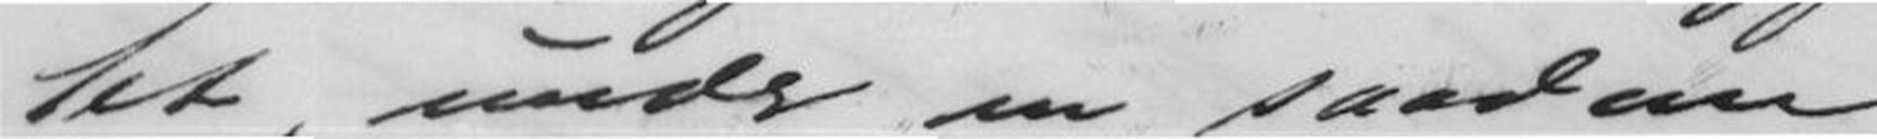let, under en saadan
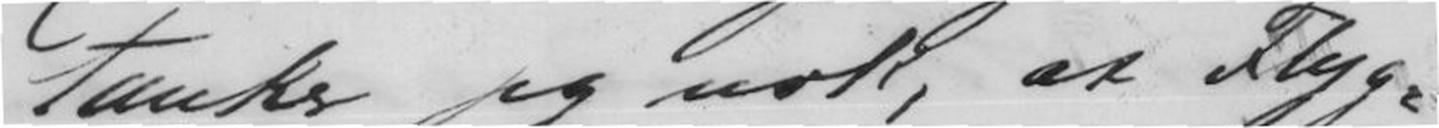tænker jeg nok, at Flyg-
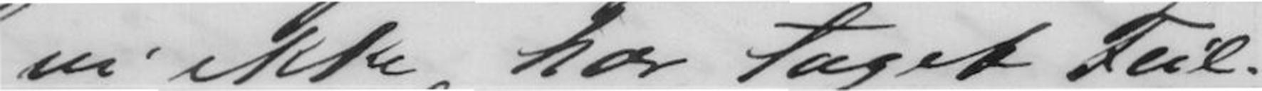vi ikke har taget Feil.
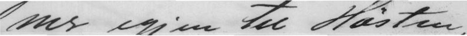mer igjen til Høsten,
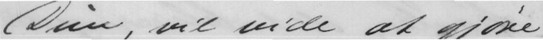Dine, vil vide at gjøre
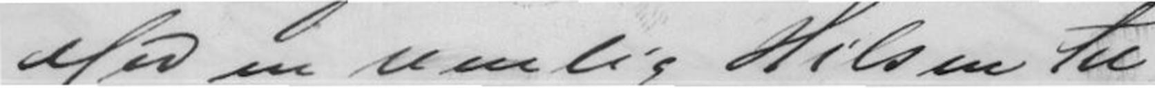Med en venlig Hilsen til
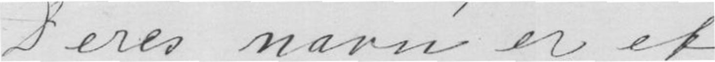Deres navn er et
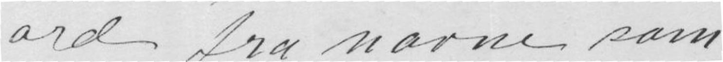ord fra navne som
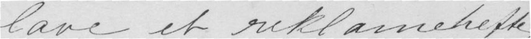lave et reklamehefte,
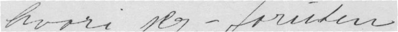hvori jeg - foruden
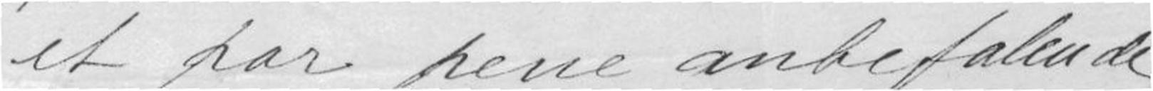et par pene anbefalende
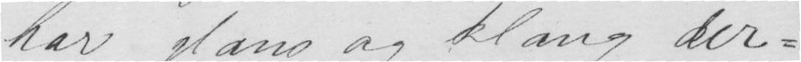har glans og klang der-
